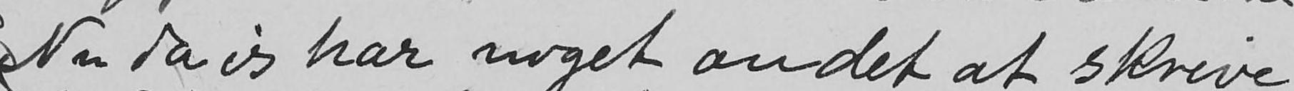Nu da jeg har noget andet at skrive
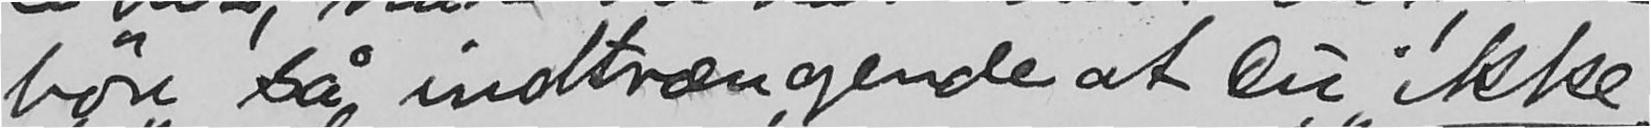bøn så indtrængende at Du ikke
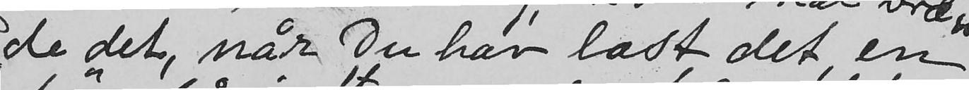de det, når Du har læst det, en
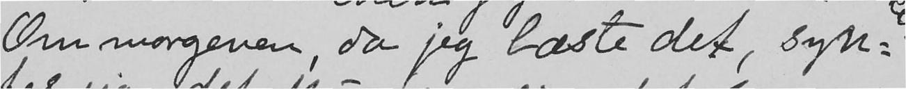Om morgenen, da jeg læste det, syn-
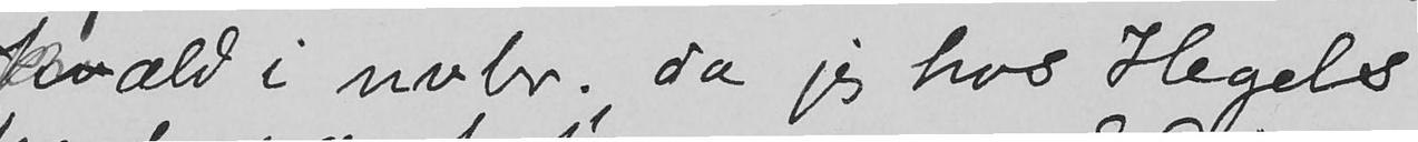kvæld i nvbr. da jeg hos Hegels
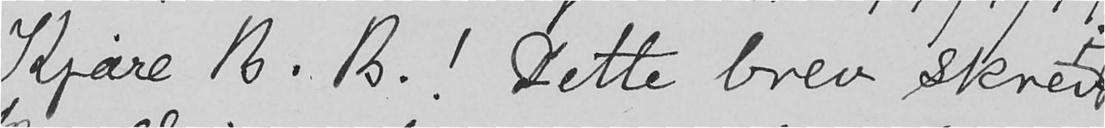Kjære B. B.! Dette brev skrev
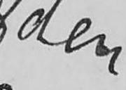den
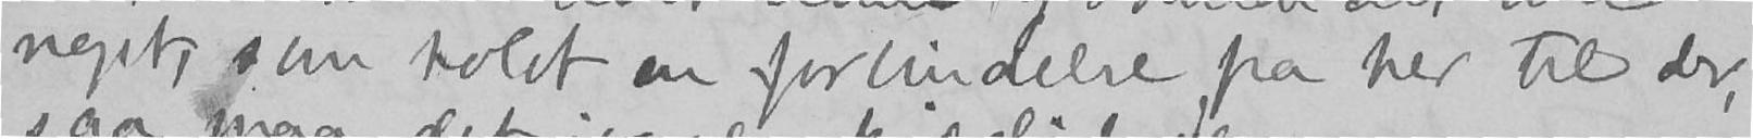noget, som holdt en forbindelse fra her til der,
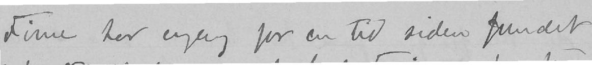Finne har engang for en tid siden fundet
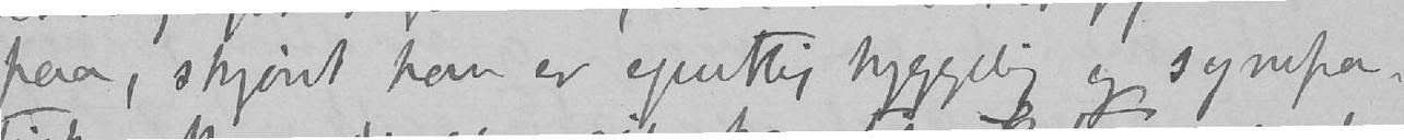paa, skjønt han er egentlig hyggelig og sympa-
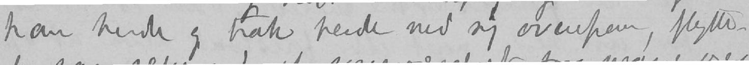han hende og trak hende med sig ovenpaa, flytte-
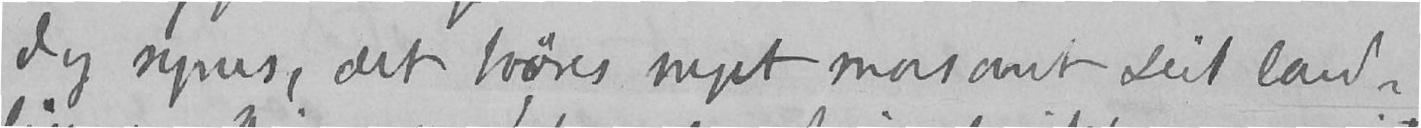Jeg synes, det høres meget morsomt Dit land-
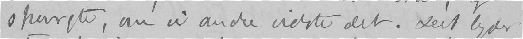spurgte, om vi andre vidste det. Det lyder
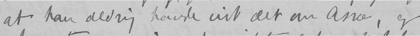at han aldrig havde vist det om Assa, og
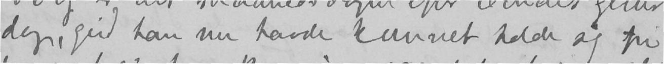dag, gid han nu havde kunnet holde sig fri
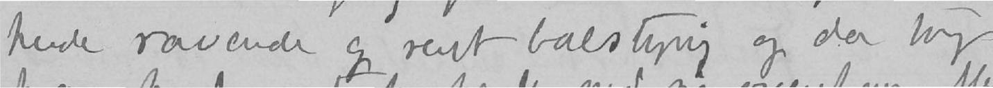hende ravende og rent balstyrig og da tog
